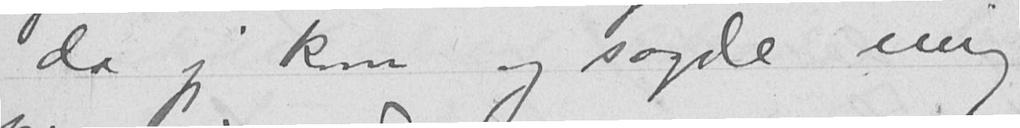da jeg kom og sagde mig
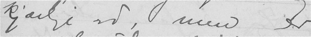kjærlige ord, men er
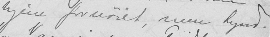hjem fornøiet, men tynd.
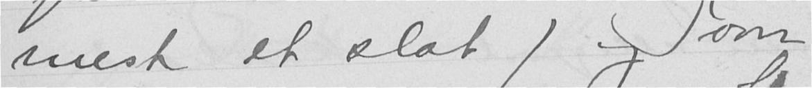mest et slot) og von
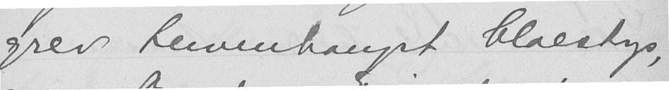grev Lewenhaupt Claestorp,
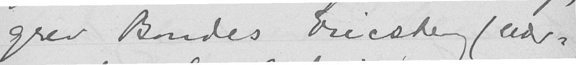grev Bondes Ericsberg (nær-
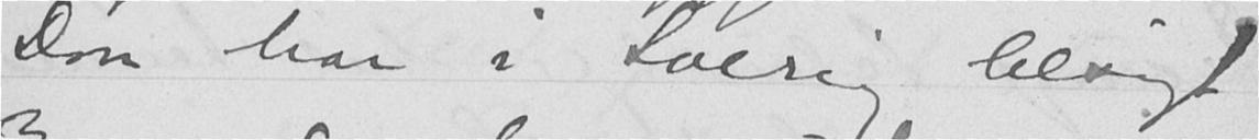Don har i Sverige besøgt
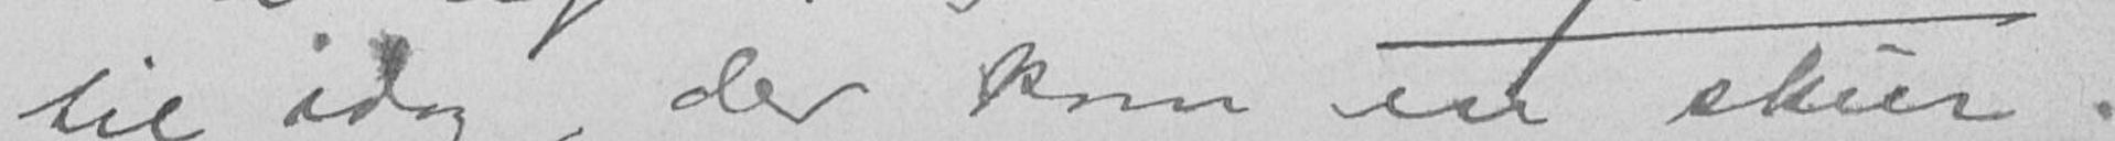til idag, der kom en skur.
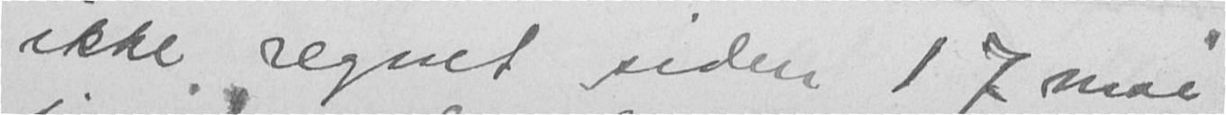ikke regnet siden 17 mai
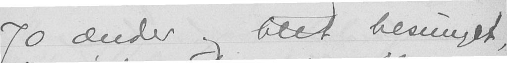70 ænder og blit besunget,
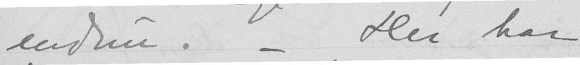endnu. - Her har
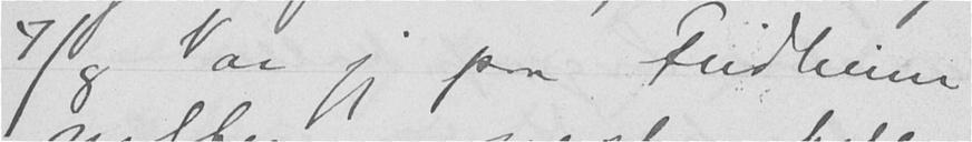7/8 Var jeg paa Fridheim
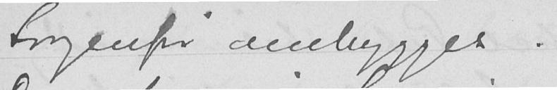Sorgenfri ombygges.
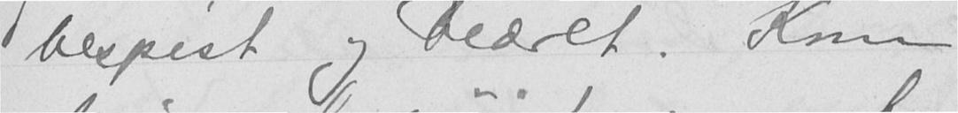bespist og beæret. Kom
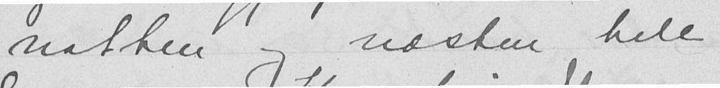natten og næsten hele
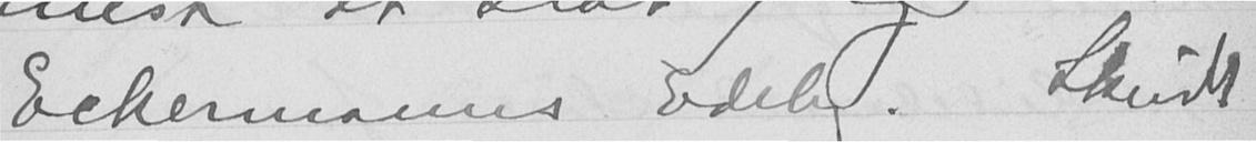Eckermanns Edeby. Skudt
